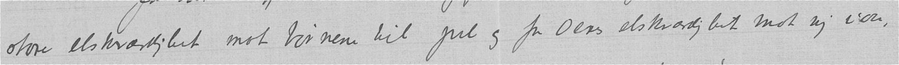store elskværdighet mot børnene til jul og for Deres elskværdighet mot mig iaar,
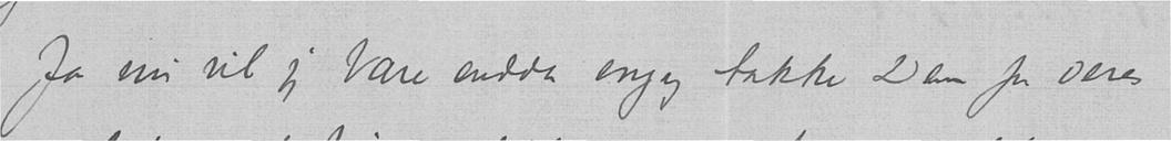Ja nu vil jeg bare endda engang takke Dem for Deres
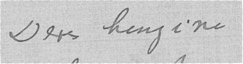Deres hengivne
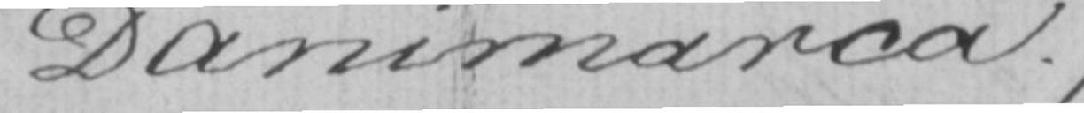Danimarca.
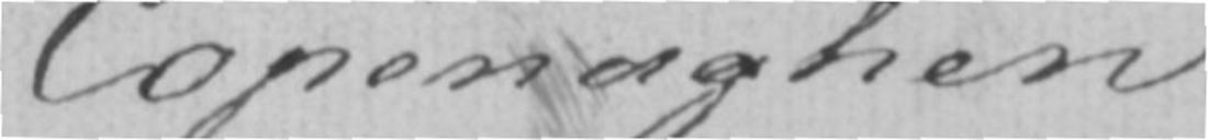Copenaghen.
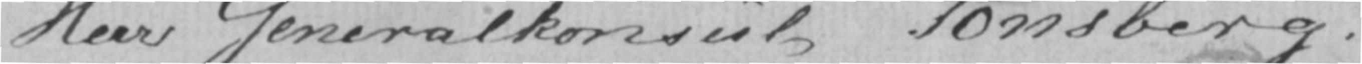Herr Generalkonsul Tønsberg.
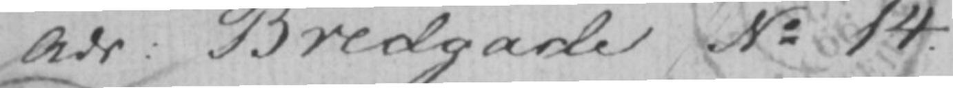Adr: Bredgade No 14.
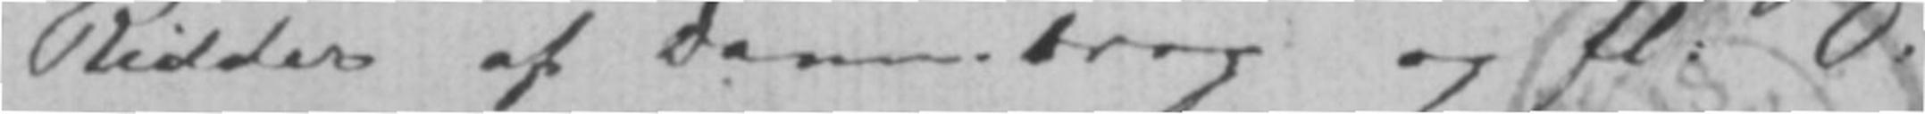Ridder af Dannebrog og fl: O:
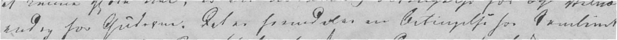endog for Guderne. Det er fremdeler en Betingelse for Samlivet
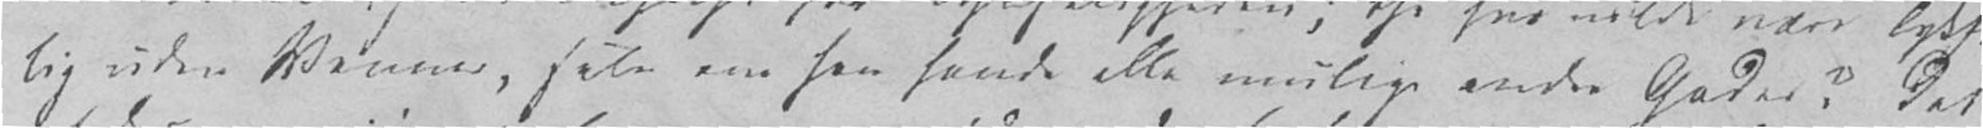lig uden Venner, selv om han havde alle mulige andre Goder? Det
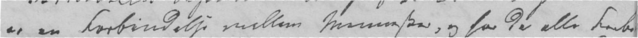er en Forbindelse mellem Mennesker, og har da alle Forbin-
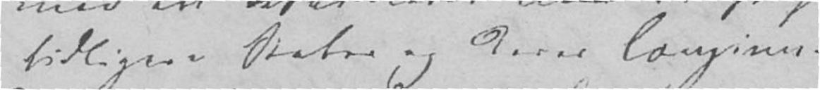tidligere Stater og deres Lovgivere
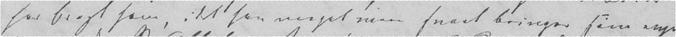har bragt ham, i det han meget mere snart bringer sine nye
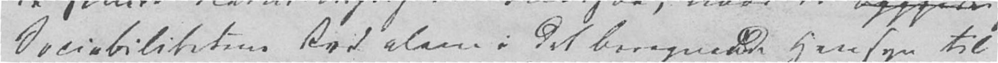Sociabilitetens Rod alene i det beregnende Hensyn til
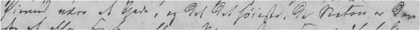Øiemed være et Gode, og det det høieste, da Staten er den
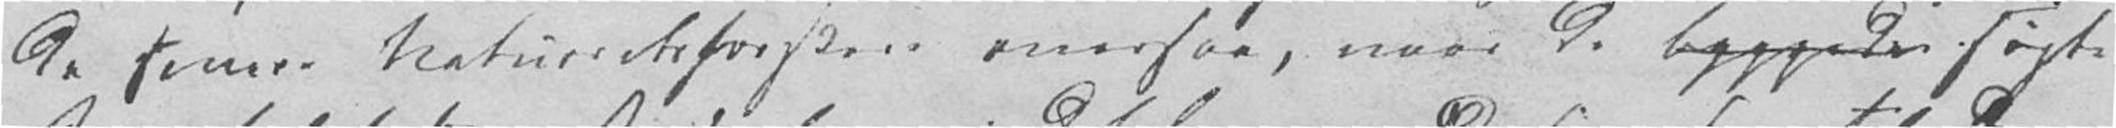de senere Naturretsforskere oversaa, naar da byggede søgte
